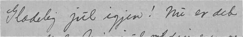Glædelig jul igjen! Nu er det
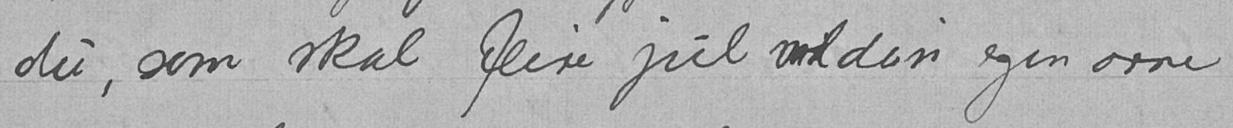du, som skal feire jul ved din egen arne
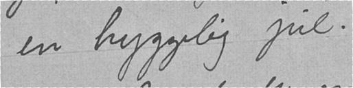en hyggelig jul.
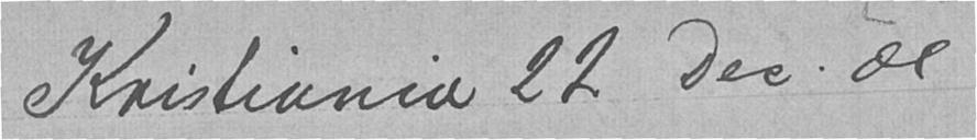Kristiania 22 Dec. 08
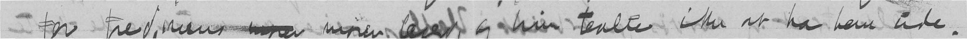for fred, mens mner moren lever, og hun tenkte ikke at ha ham ude.
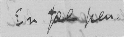En fæl pen.
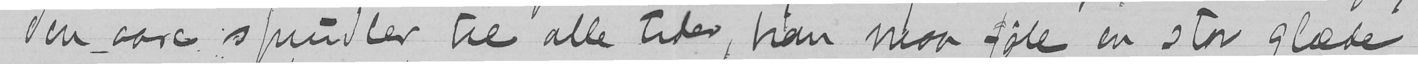den aare sprudler til alle tider, han maa føle en stor glæde
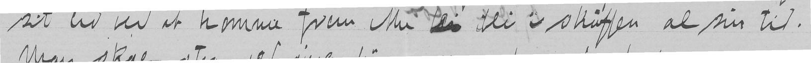sit liv ved at komme frem ikke tli bli i skuffen al sin tid.
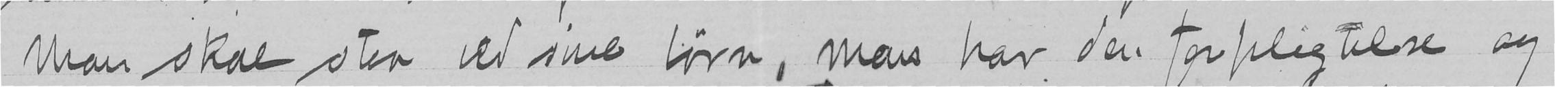Man skal staa ved sine børn, man har den forpligtelse og
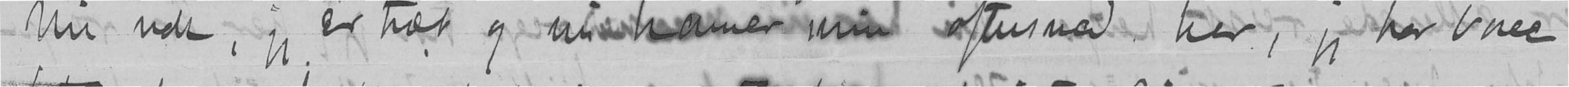Nu nok, jeg er træt og nu kommer min aftenmad her, jeg har bare
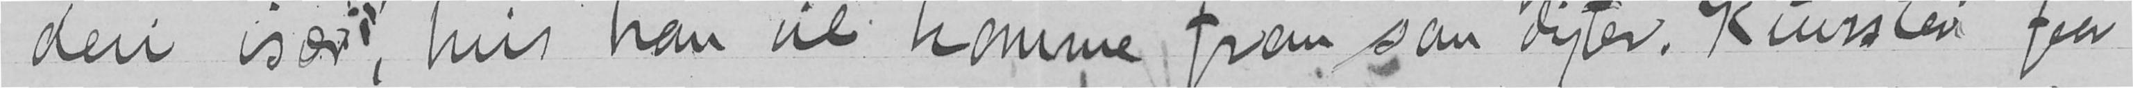deri især, hvis han vil komme frem som digter. Kunsten faar
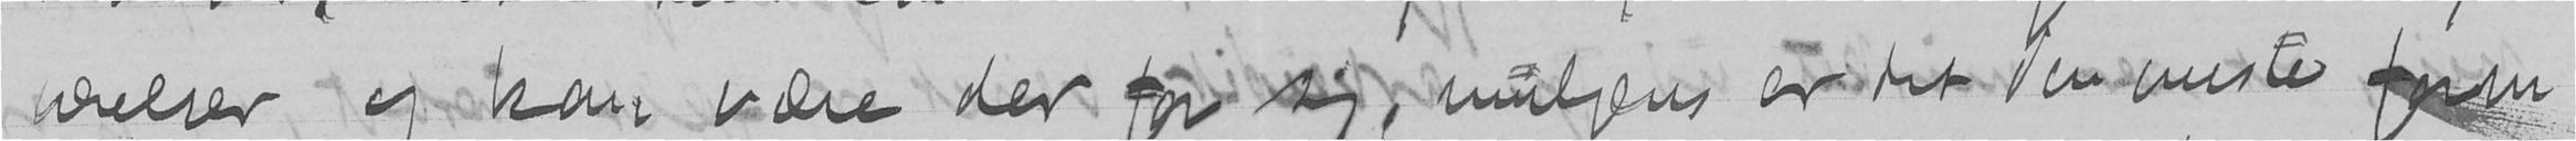værelser og kan være der for sig, muligens er det den eneste form
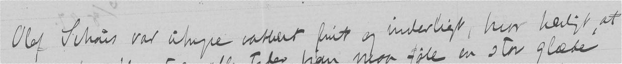Olaf Schous var uhyre vakkert frit og inderligt, hvor herligt, at
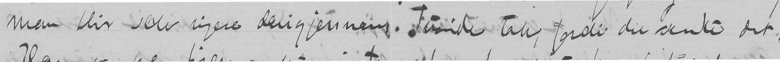man blir selv rigere derigjennem. Tusinde tak, fordi du sendte det.
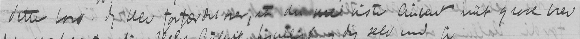dette bord. Jeg blev forfærdet over, at du med viste Aubert mit grove brev
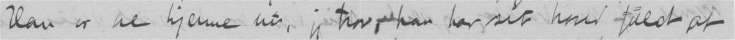Han er vel hjemme nu, jeg tror, han har sit hoved fuldt af
